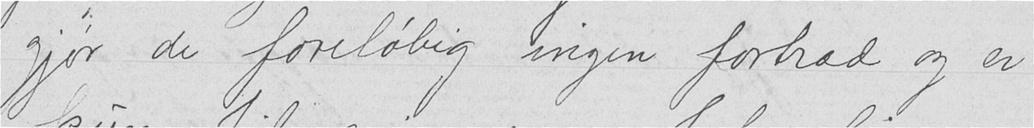gjør de foreløbig ingen fortræd og er
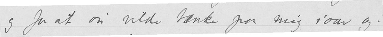og for at du vilde tænke paa mig iaar og.
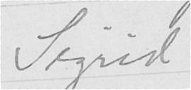Sigrid.
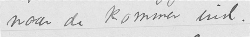naar de kommer ind.
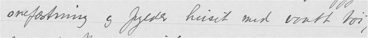snefæstning og fylder huset med vaatt tøi,
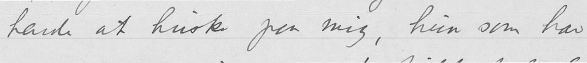hende at huske paa mig, hun som har
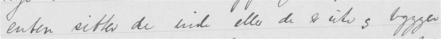enten sitter de inde eller de er ute og bygger
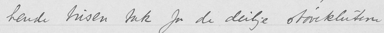hende tusen tak for de deilige støveklutene
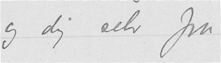og dig selv fra
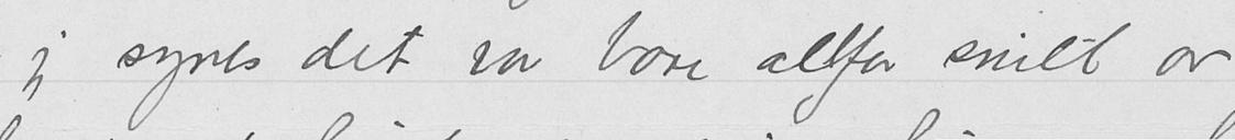- jeg synes det var bare allfor snilt av
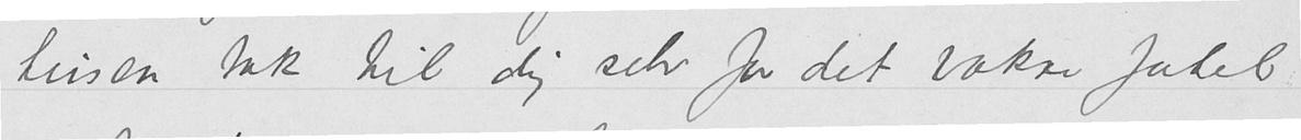tusen tak til dig selv for det vakre fatet
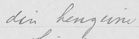din hengivne
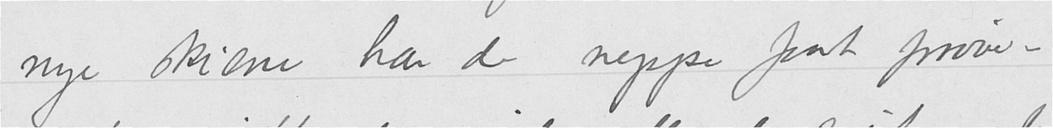nye skiene har de neppe faat prøve –
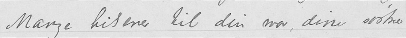Mange hilsener til din mor, dine søstre
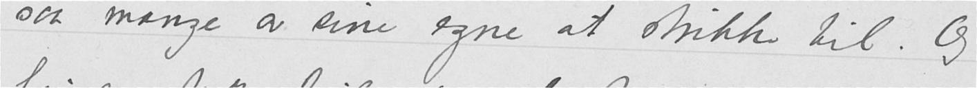saa mange av sine egne at strikke til. Og
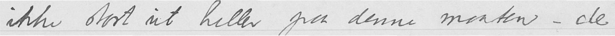ikke stort ut heller paa denne maaten - de
